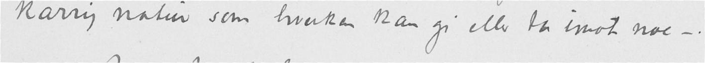karrig natur som hverken kan gi eller ta imot noe –.
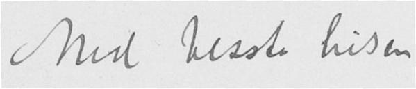Med besste hilsen
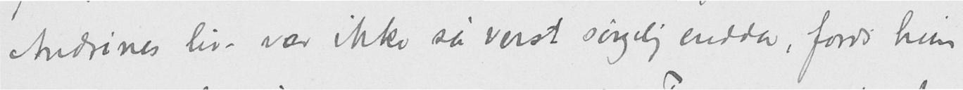Andrines liv var ikke så verst sørgelig endda, fordi hun
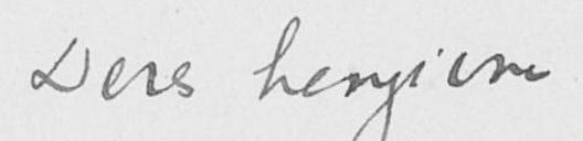Deres hengivne
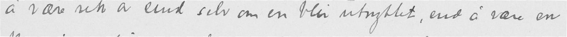å være rik av sind selv om en blir utnyttet, end å være en
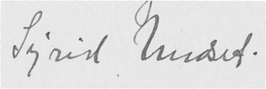Sigrid Undset.
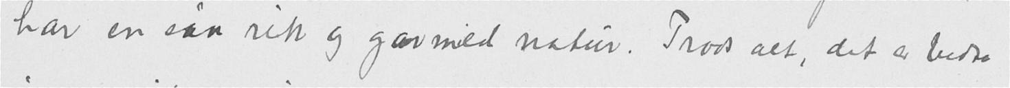har en saa rik og gavmild natur. Trods alt, det er bedre
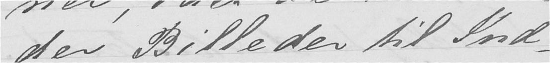der Billeder til Ind-
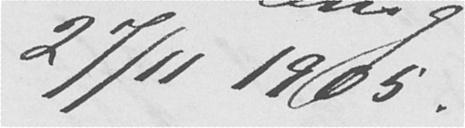27/11 1905.
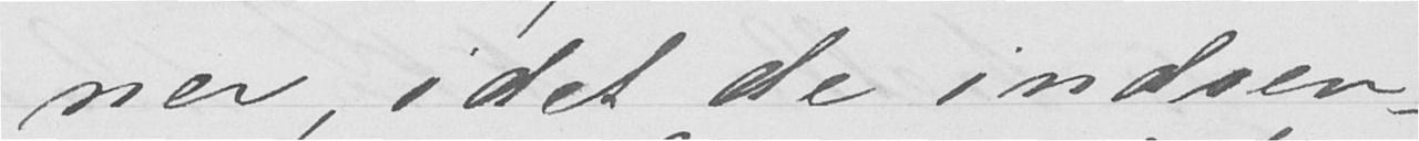ner, i det de indsen-
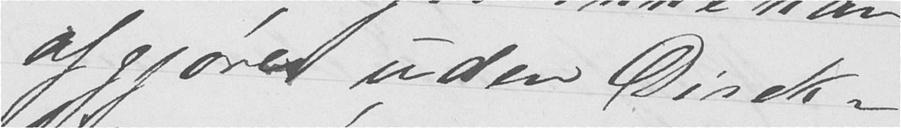afgjøres uden Direk-
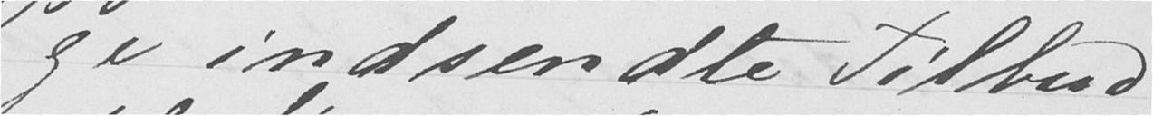ge indsendte Tilbud
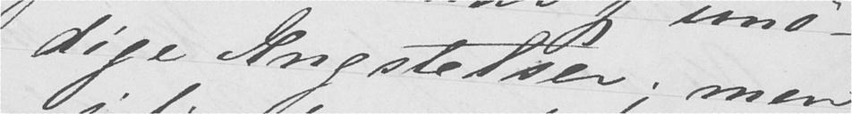dige Ængstelser; men
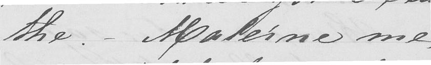the. – Malerne me-
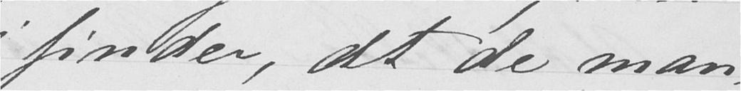jeg finder, at de man-
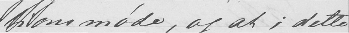tionsmøde, og at i dette
